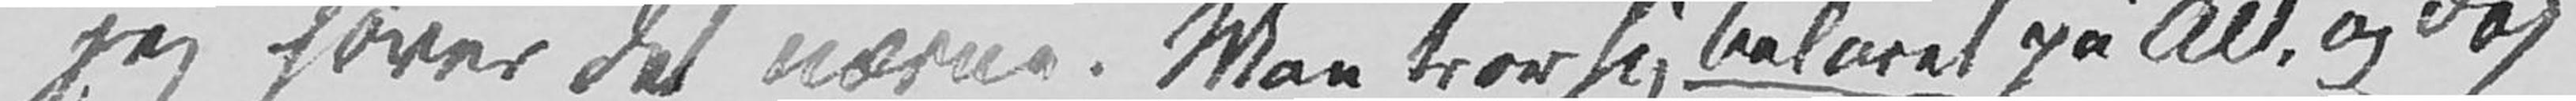jeg hører det nævne. Man tror sig belæret på Alt, og dog
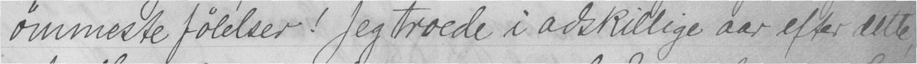ømmeste følelser! Jeg troede i adskillige aar efter dette
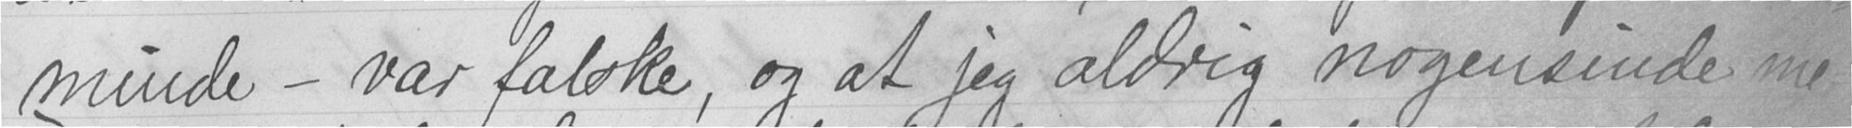minde - var falske, og at jeg aldrig nogensinde me-
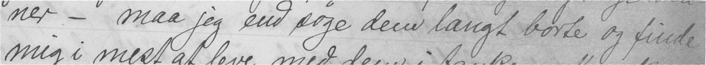ner - maa jeg end søge dem langt borte og finde
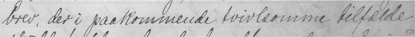brev, der i paakommende tvivlsomme tilfælde
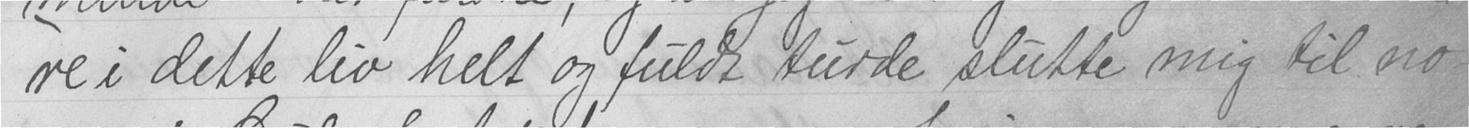re i dette liv helt og fuldt turde slutte mig til no-
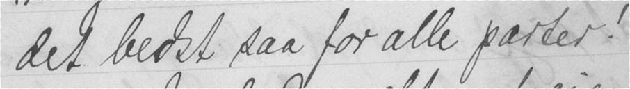det bedst saa for alle parter!
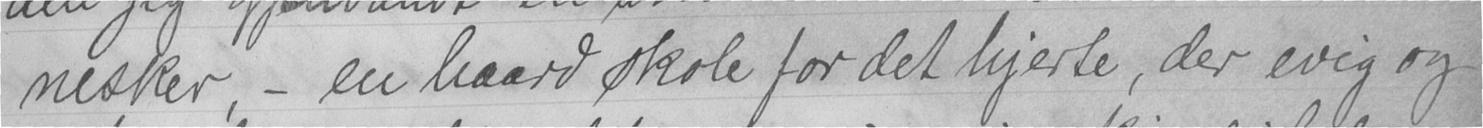nesker, - en haard skole for det hjerte, der evig og
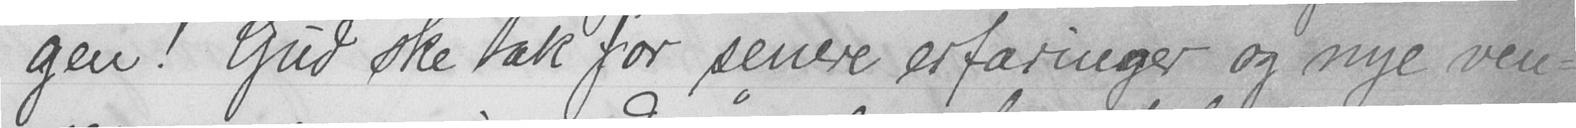gen! Gud ske tak for senere erfaringer og nye ven-
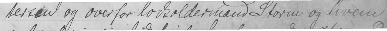tersen og overfor lodsoldermand Storm og hvem
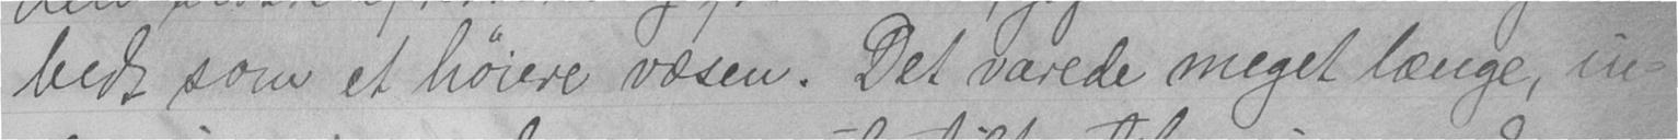bedt som et høiere væsen. Det varede meget længe, in-
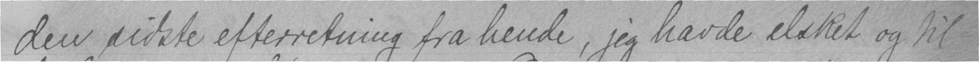den sidste efterretning fra hende, jeg havde elsket og til-
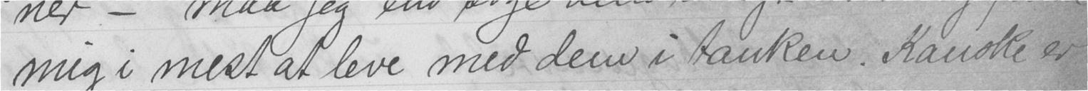mig i mest at leve med dem i tanken. Kanske er
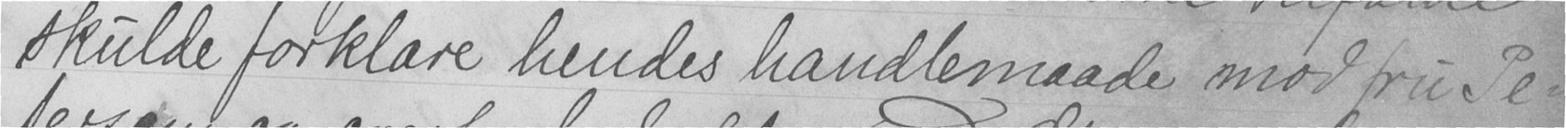skulde forklare hendes handlemaade mod fru Pe-
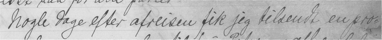Nogle dage efter afreisen fik jeg tilsendt en pro-
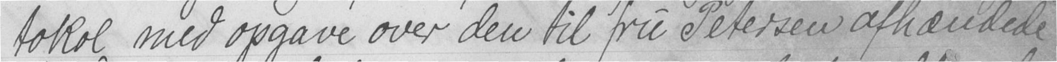tokol med opgave over den til fru Petersen afhændede
